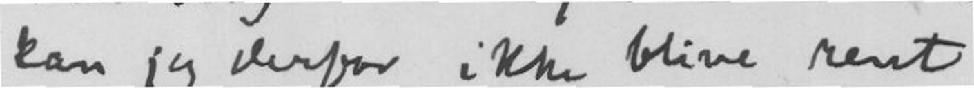kan jeg derfor ikke blive rent
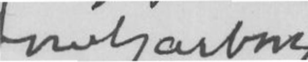Arne Garborg.
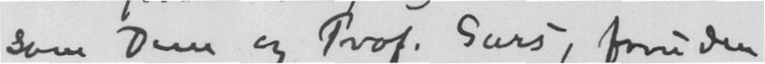som Dem og Prof. Sars, foruden
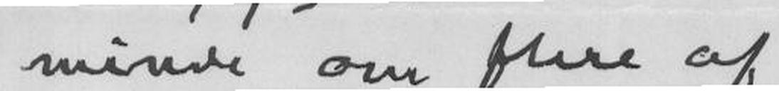minde om flere af
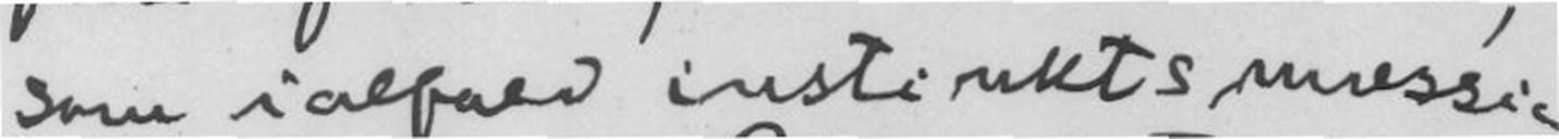som ialfald instinktsmæssig
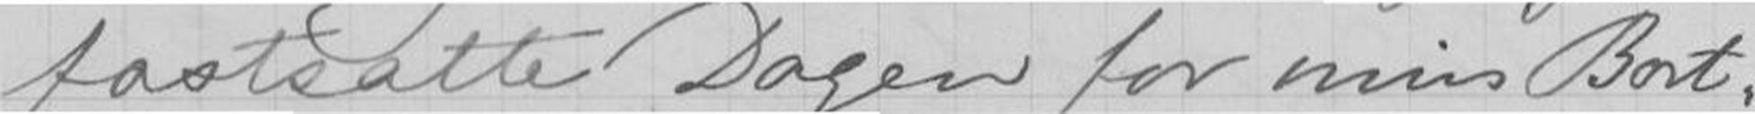fastsatte Dagen for min Bort-
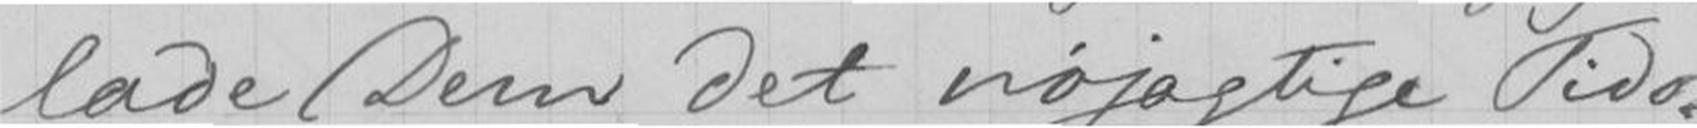lade Dem det nøjagtige Tids-
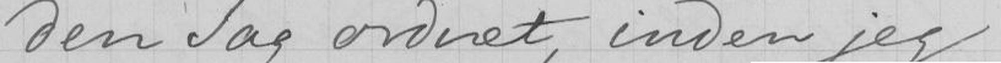den Sag ordnet, inden jeg
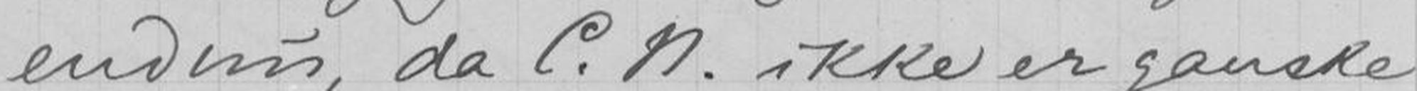endnu, da C. N. ikke er ganske
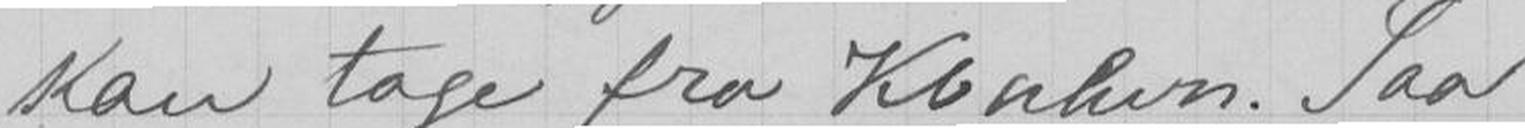kan tage fra Kbnhvn. Saa
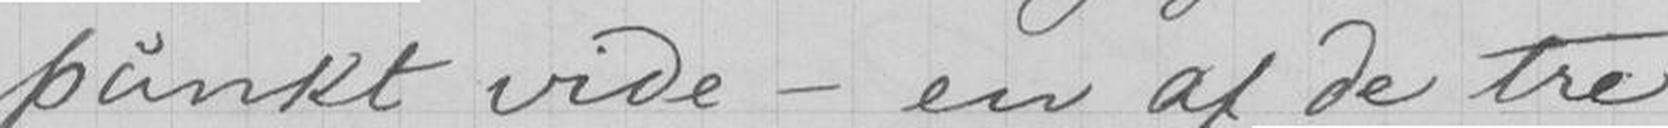punkt vide - en af de tre
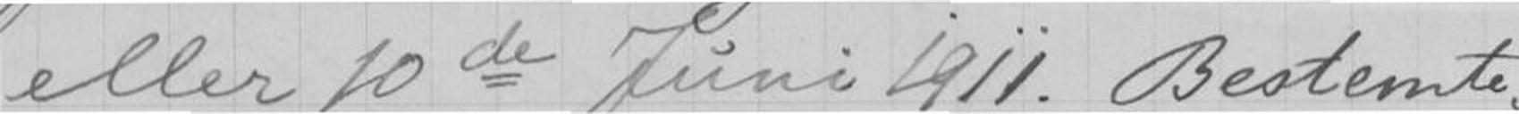eller 10de Juni 1911. Bestemte-
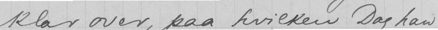klar over, paa hvilken Dag han
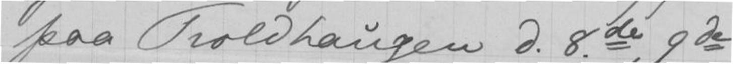paa Troldhaugen d. 8de, 9de
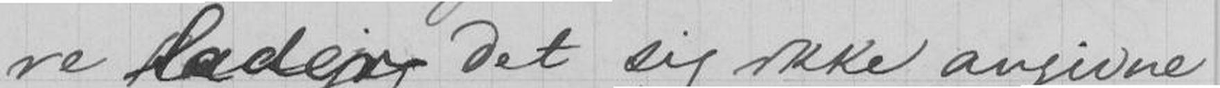re lader det sig ikke angivne
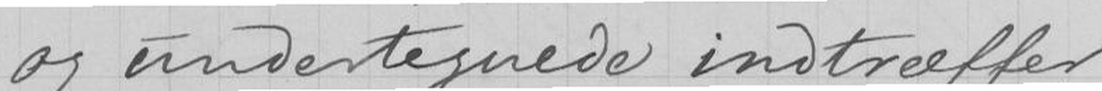og undertegnede indtræffer
